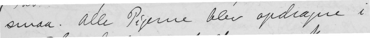smaa. Alle Pigerne blev opdragne i
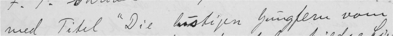med Titel "Die lustigen Gungfern vom
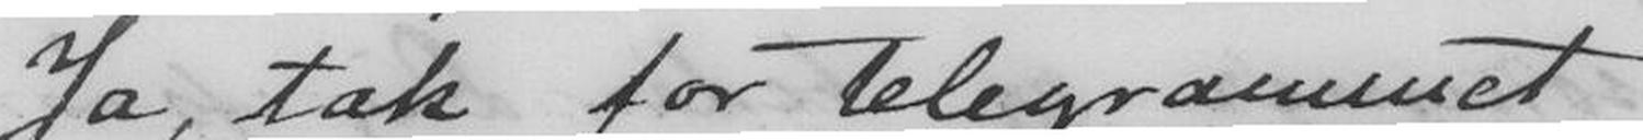Ja, tak for telegrammet
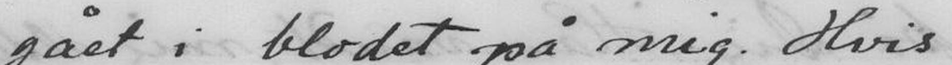gået i blodet på mig. Hvis
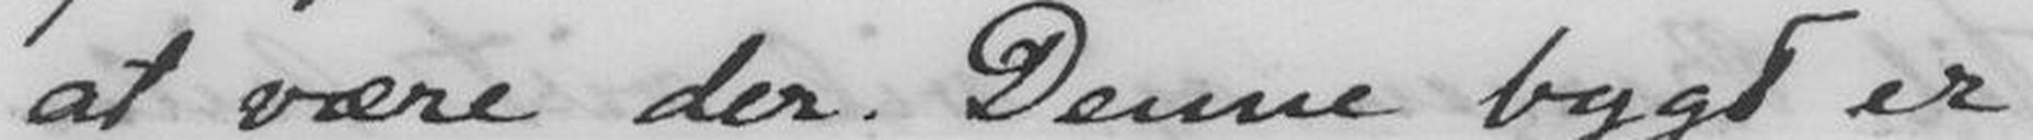at være der. Denne bygd er
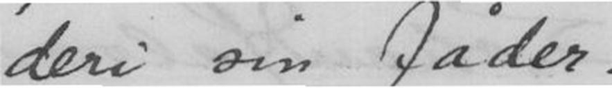deri sin Fader.
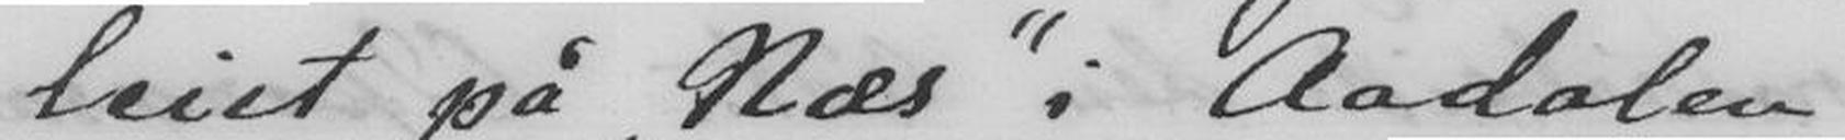leiet på Næs i Aadalen
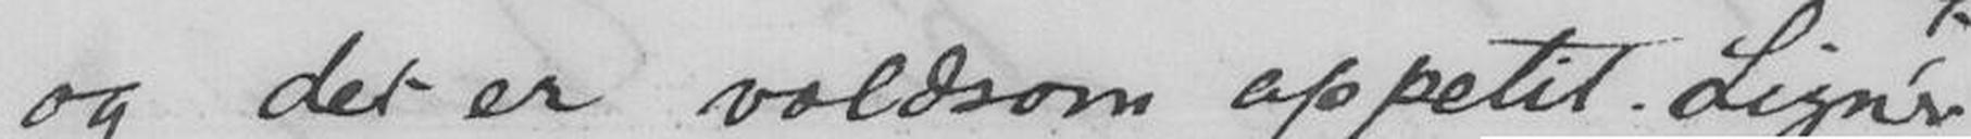og det er voldsom appetit. Ligner
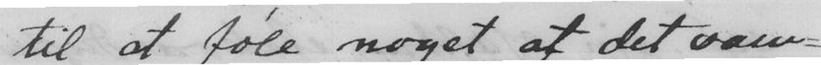tilat føle noget af det sam-
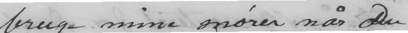bruge mine snører når Du
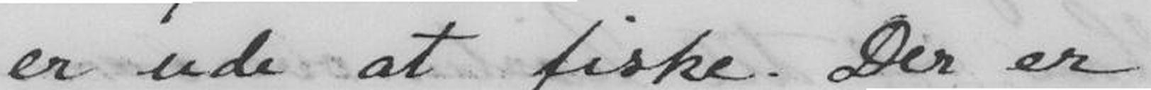er ude at fiske. Der er
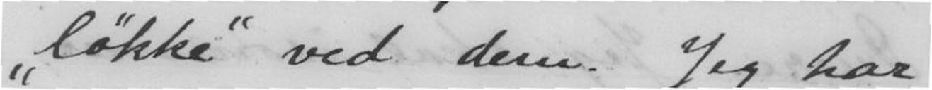løkke ved dem. Jeg har
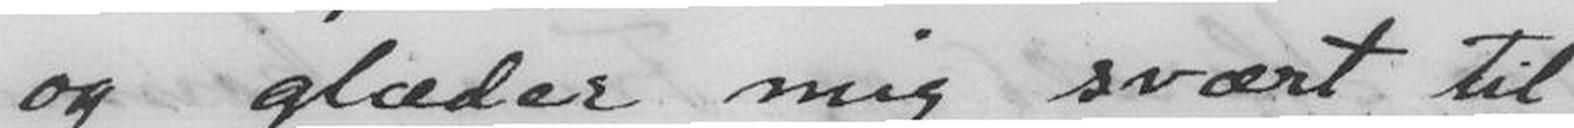og glæder mig svært til
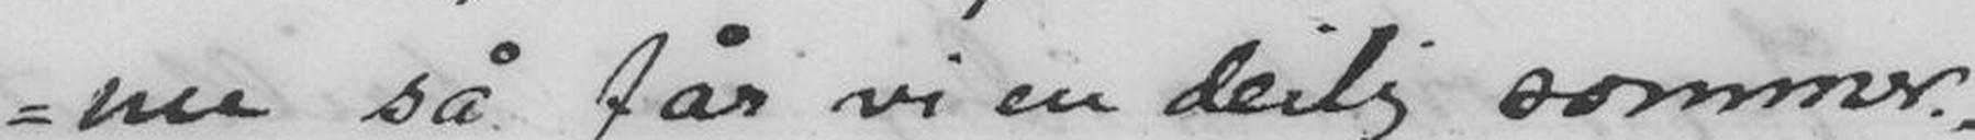me så får vi en deilig sommer. -
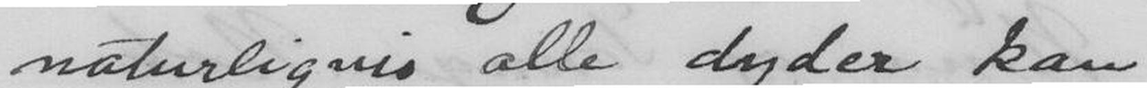naturligvis alle dyder kan
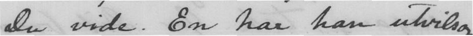du vide. En har han utvilsomt
